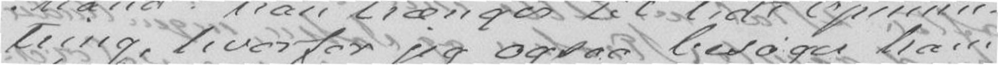tring, hvorfor jeg ogsaa besøger ham
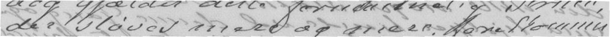der sløves mere og mere forekommer
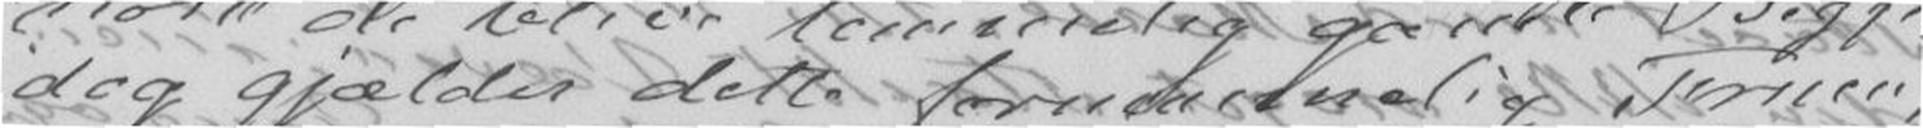dog gjælder dette fornemmelig Fruen,
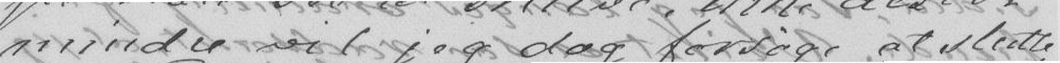mindre vil jeg dog forsøge at slutte
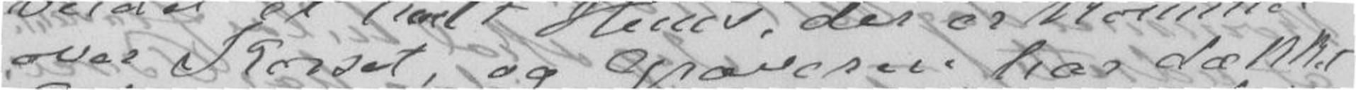over Korset, og Graveren har dækket
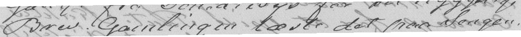Brev. Gamlingen læste det paa Sengen,
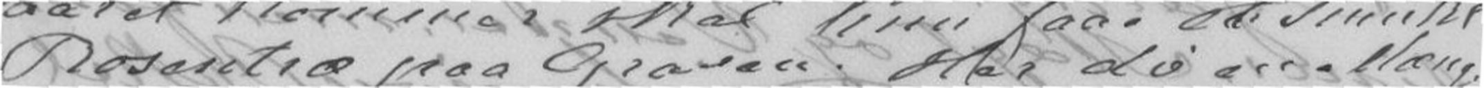Rosentræ paa Graven. Her dø en Mæng-
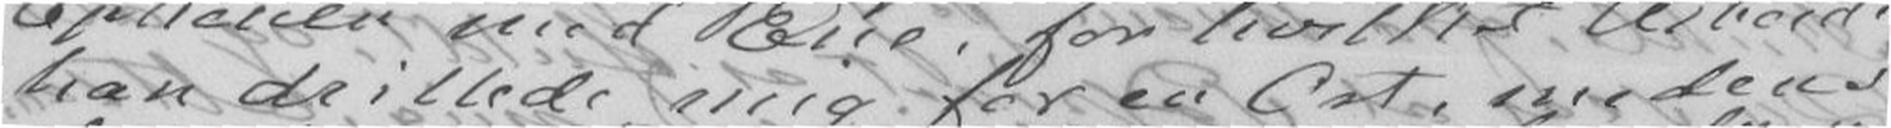han drillede mig for en Ort, medens
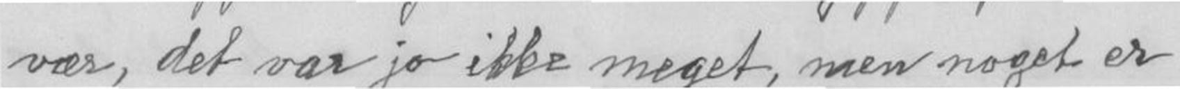vær, det var jo ikke meget, men noget er
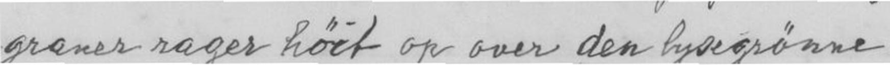graner rager høit op over den lysegrønne
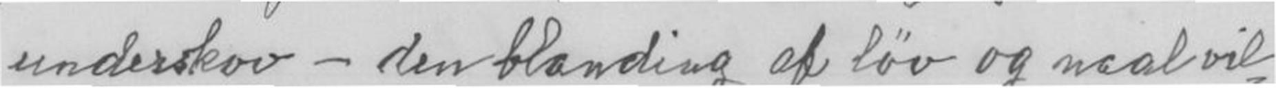underskov - den blanding af løv og maal vil-
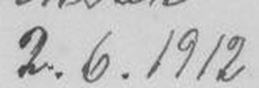2.6.1912
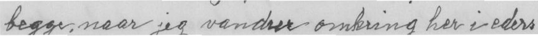begge, naar jeg vandrer omkring her i eders
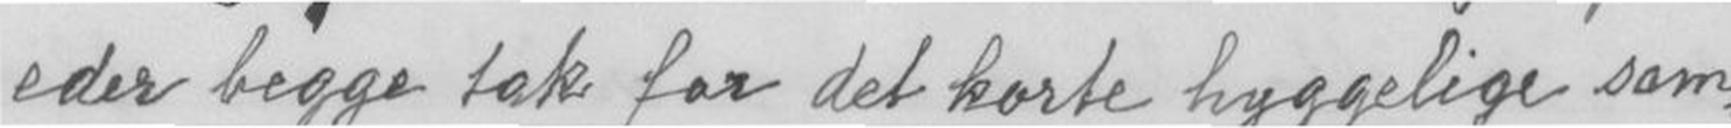eder begge tak for det korte hyggelige sam-
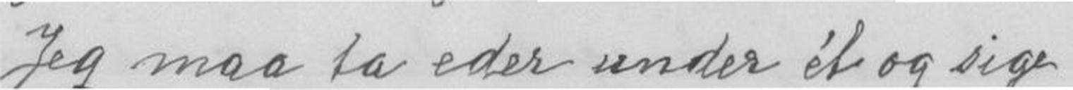Jeg maa ta eder under et og sige
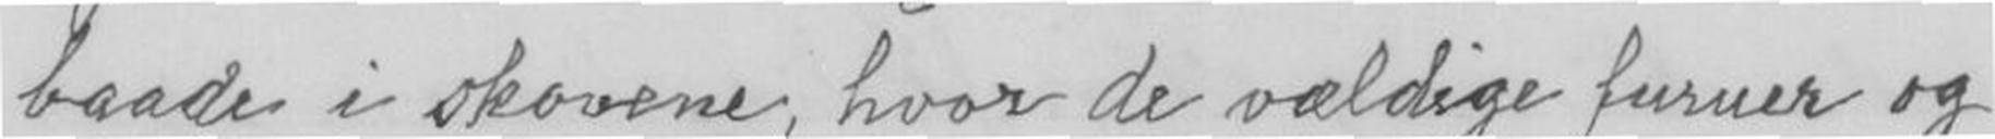baade i skovene, hvor de vældige furer og
